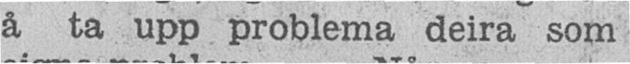å ta upp problema deira som
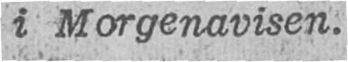i Morgenavisen.
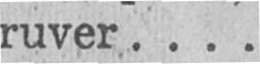ruver....
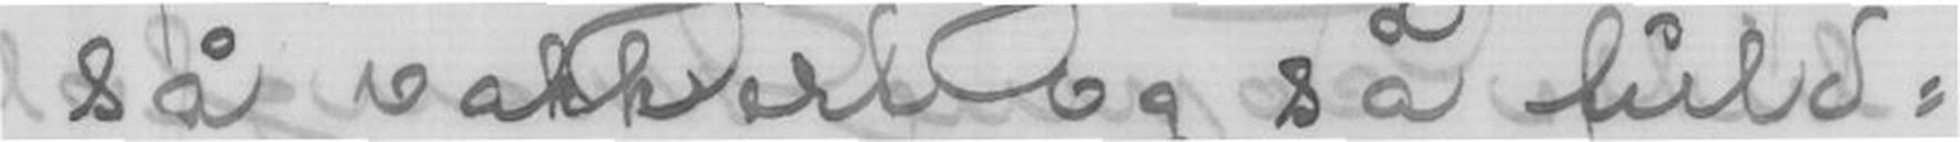så vakkert og så fuld-
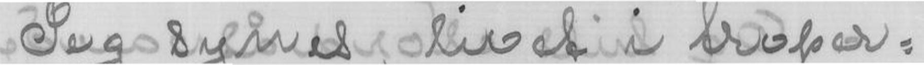Jeg synes, livet i troper-
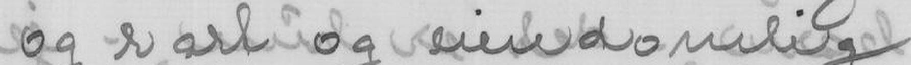og rart og eiendomlig
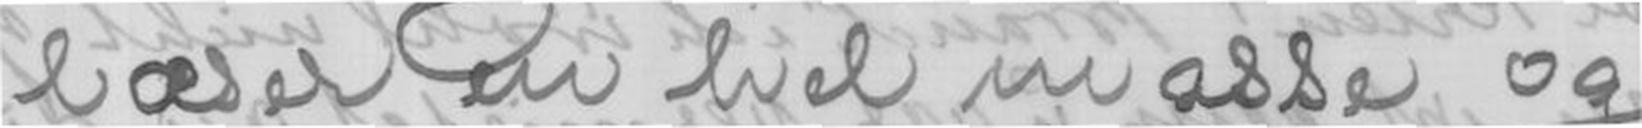læser en hel masse og
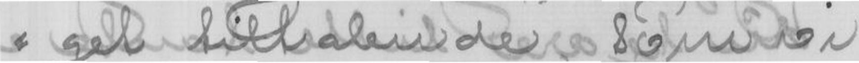ger tiltalende, som vi
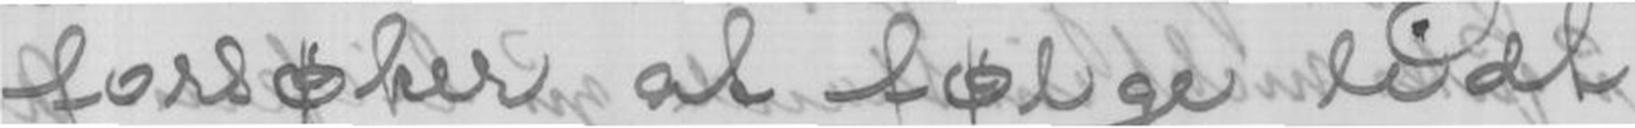forsøker at følge lidt
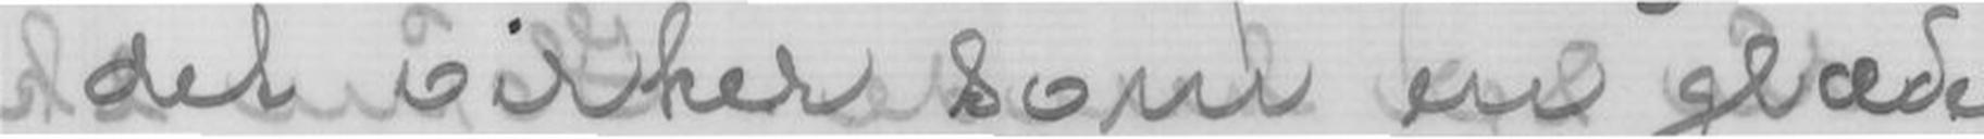det virker som en glæde.
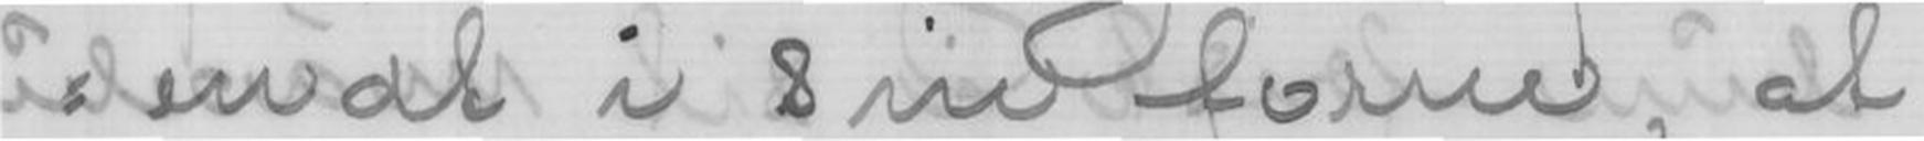endt i sin form, at
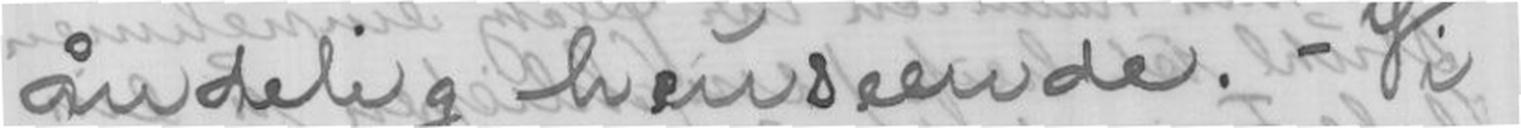åndelig henseende. - Vi
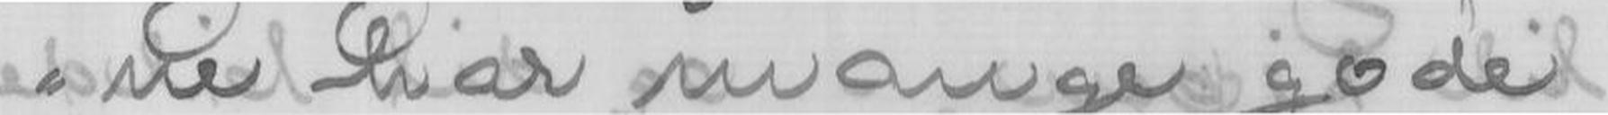ne har mange gode
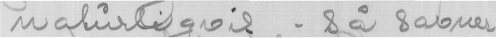- naturligvis, - så savner
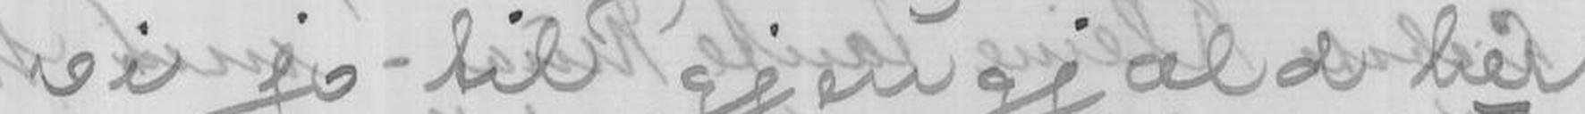vi jo til gjengjæld her
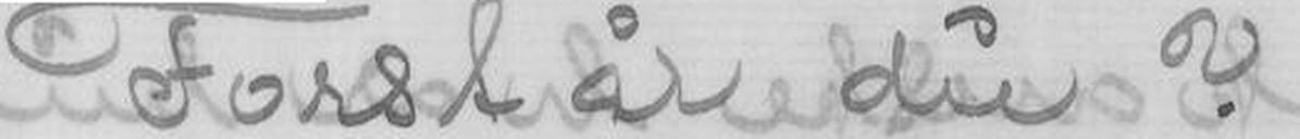- Fostår du?
